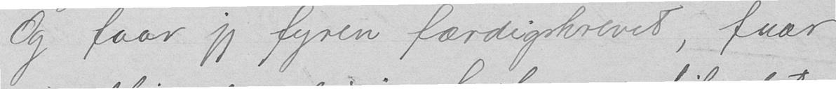Og faar jeg fyren færdigskrevet, faar
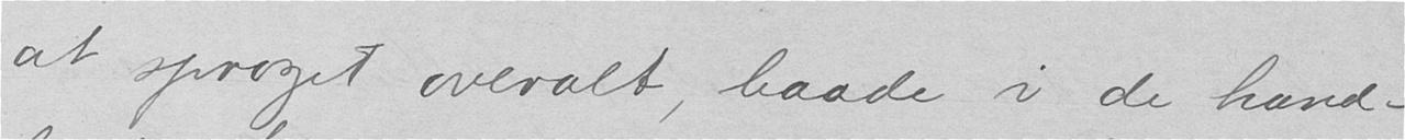at sproget overalt, baade i de hand-
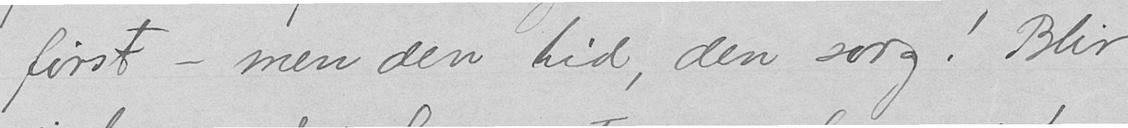først - men den tid, den sorg! Blir
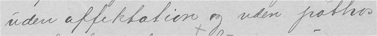uden affektation, og uden pathos
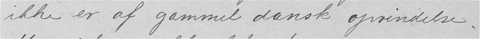ikke er af gammel dansk oprindelse.
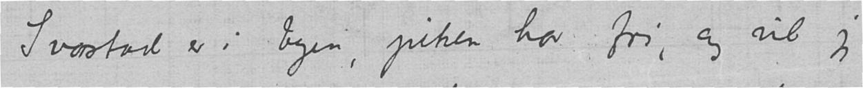Svarstad er i byen, piken har fri, og vil jeg
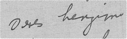Deres hengivne
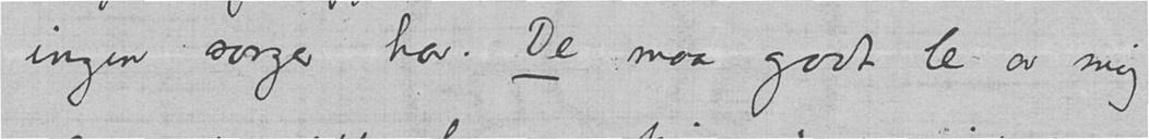ingen sorger har. De maa godt le av mig!
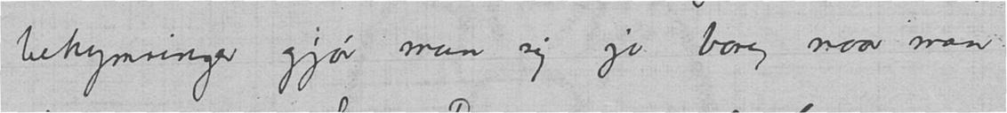bekymringer gjør man sig jo bare, naar man:
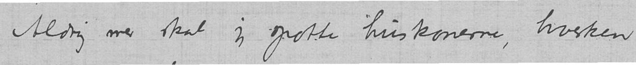Aldrig mer skal jeg spotte huskonerne, hverken
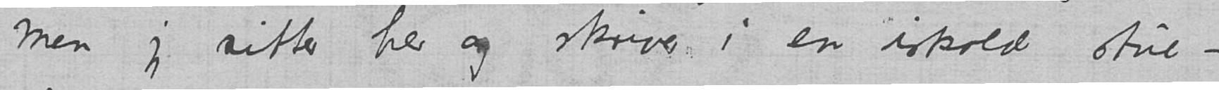Men jeg sitter her og skriver i en iskold stue -
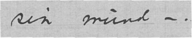sin mund -.
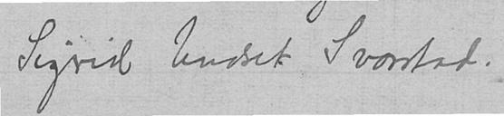Sigrid Undset Svarstad.
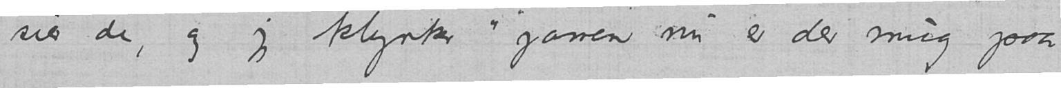sier de, og jeg klynker "jamen nu er der mug paa
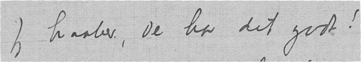Jeg haaber, De har det godt!
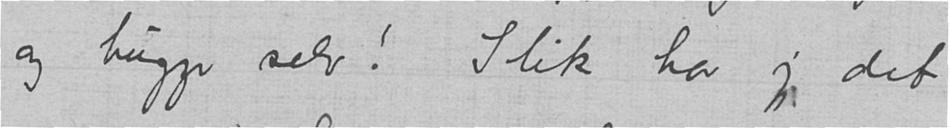og hugge selv! Slik har jeg det.
